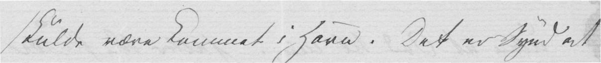skulde være kommet i Havn. Det er Synd at
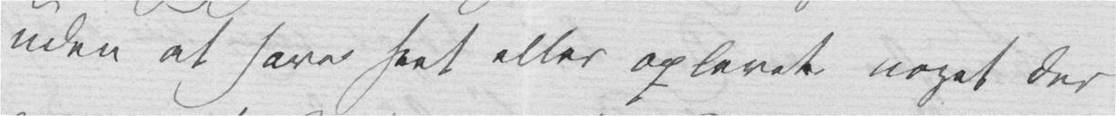uden at have seet eller oplevet noget der
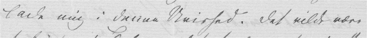lade mig i denne Uvished. Det vilde være
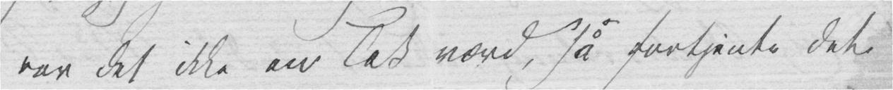var det ikke en Tak værd, så fortjente det
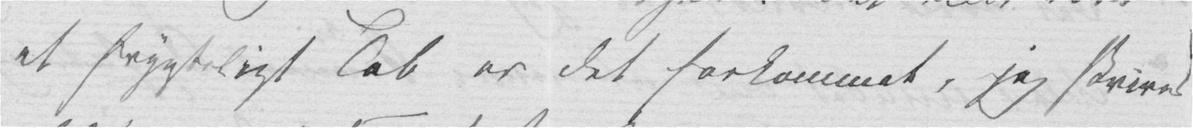et frygteligt Tab er det forkommet, jeg skriver
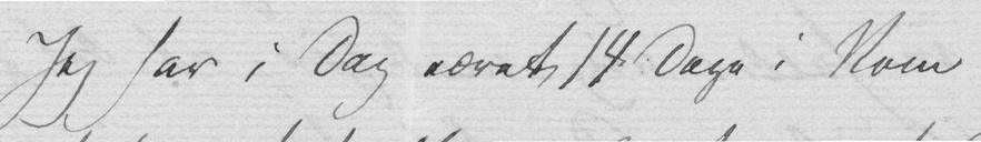Jeg har i Dag været 14 Dage i Rom
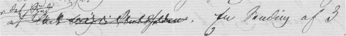at lade mig i Uvisheden. En Sending af 3
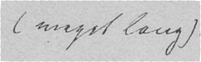(meget lang).
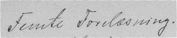Femte Forelæsning.
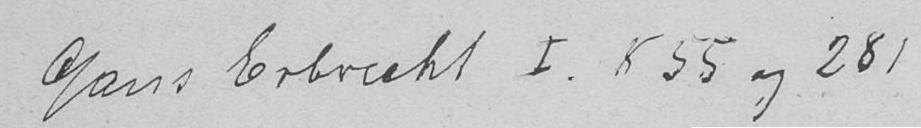Gans Erbrecht I § 55 og 281
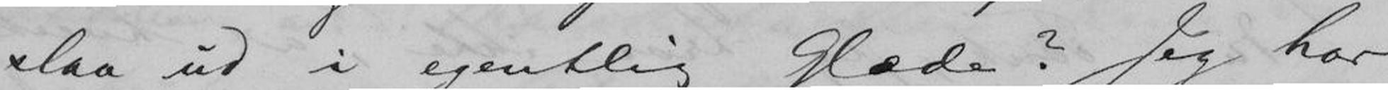slaa ud i egentlig Glæde? Jeg har
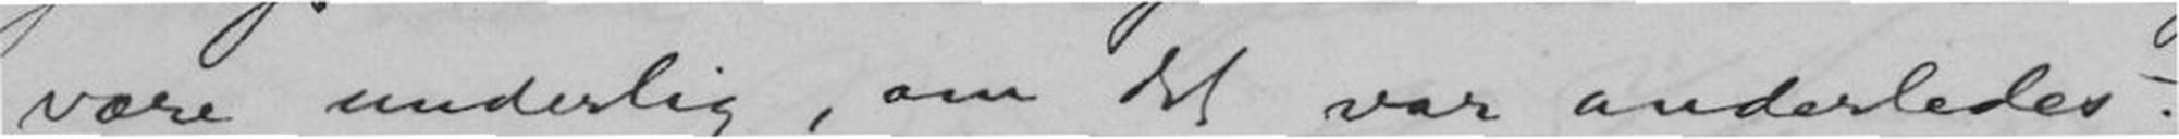være underligt, om det var anderledes.
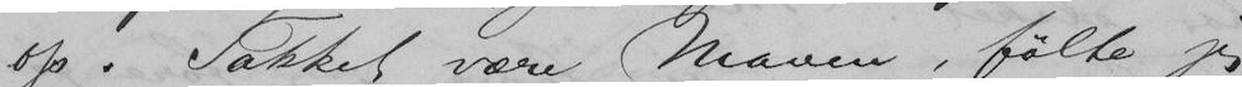op. Takket være Maven, følte jeg
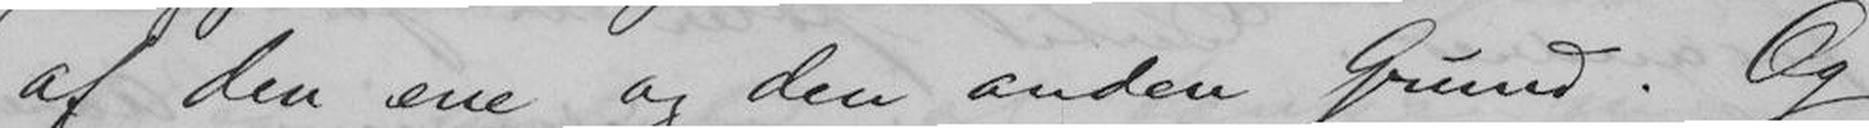af den ene og den anden Grund. Og
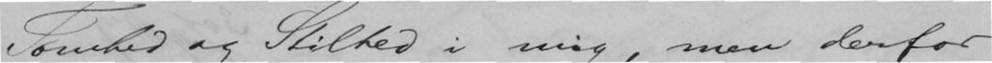Tomhed og Stilhed i mig, men derfor
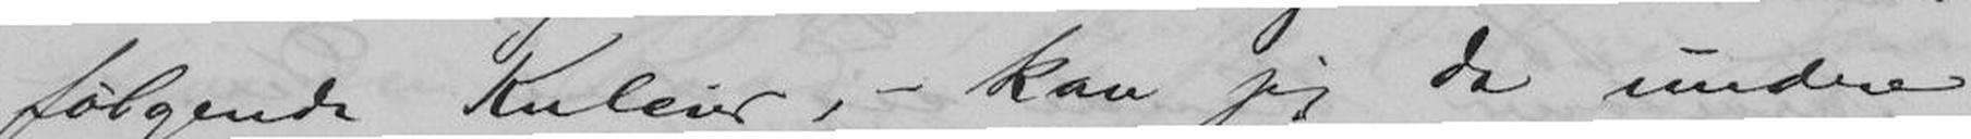følgende Kuleur, - kan jeg da undre
